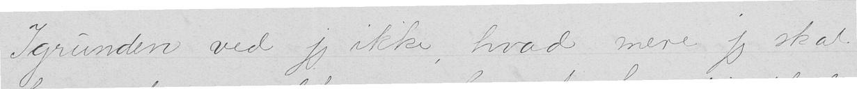Igrunden ved jeg ikke, hvad mere jeg skal
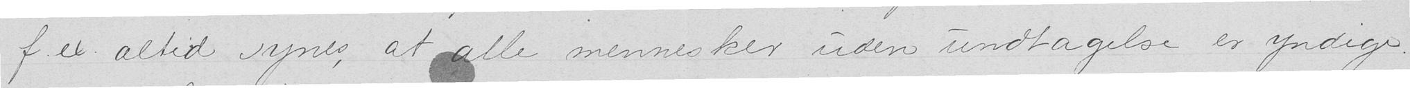f.ex. altid synes, at alle mennesker uden undtagelse er yndige.
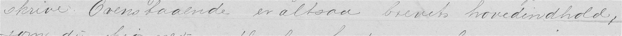skrive. Ovenstaaende er altsaa brevets hovedindhold,
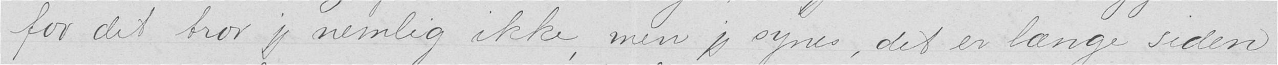for det tror jeg nemlig ikke, men jeg synes, det er længe siden
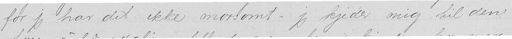for jeg har det ikke morsomt - jeg kjeder mig til den
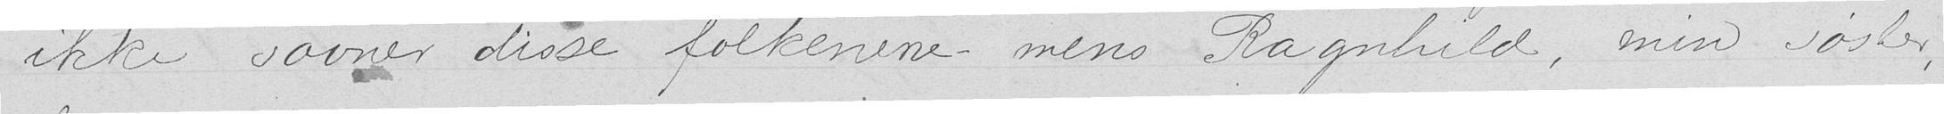ikke savner disse folkenene - mens Ragnhild, min søster,
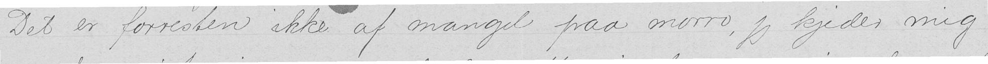Det er forresten ikke af mangel paa morro, jeg kjeder mig
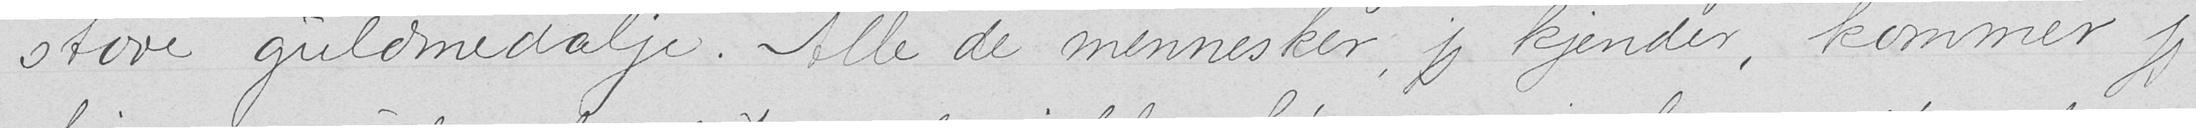store guldmedalje. Alle de mennesker, jeg kjender, kommer jeg
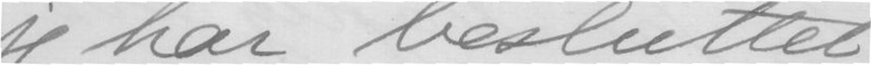jeg har besluttet
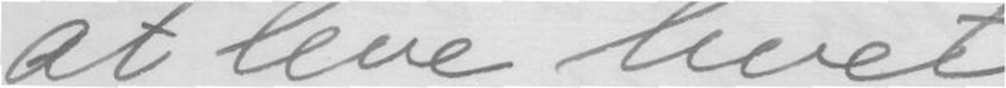at leve livet
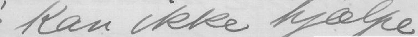jeg kan ikke hjælpe
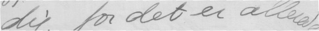dig, for det er alleræde
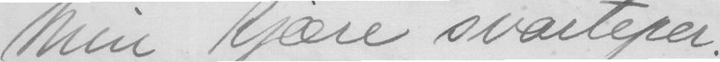Min kjære svarteper!
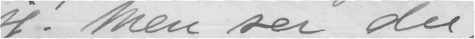jeg! Men ser du,
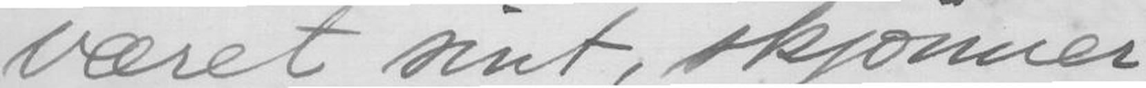været sint, skjønner
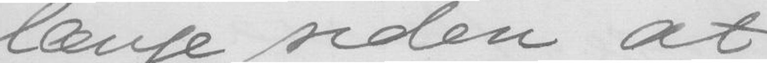længe siden at
